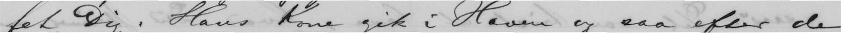fet Dig. Hans Kone gik i Haven og saa efter de
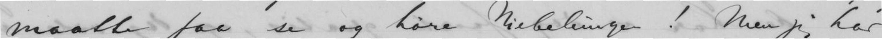maatte faa se og høre Niebelungen! Men jeg har
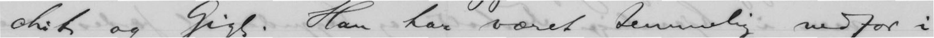chit og Gigt. Han har været temmelig nedfor i
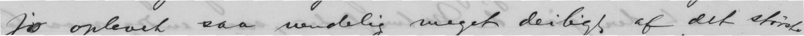jo oplevet saa uendelig meget deiligt af det største,
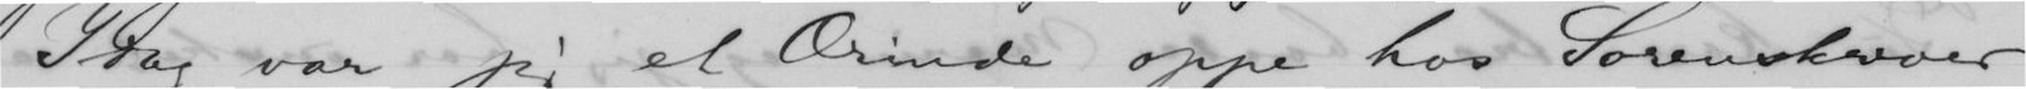Idag var jeg et Ærinde oppe hos Sorenskriver
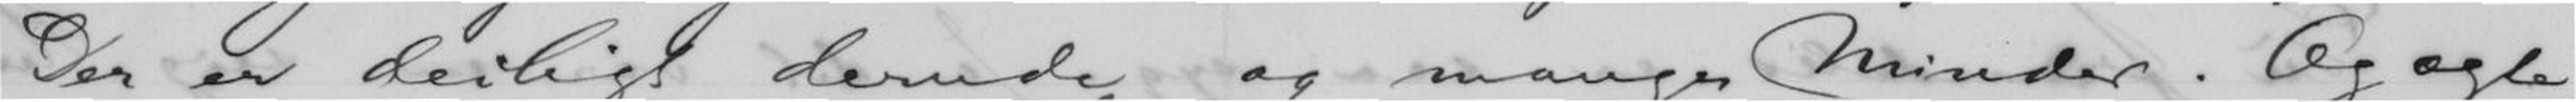Der er deiligt derude og mange Minder. Og ægte
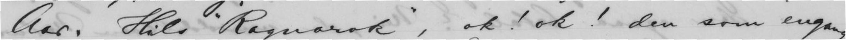Aar. Hils "Ragnarok", ok! ok! den som engang
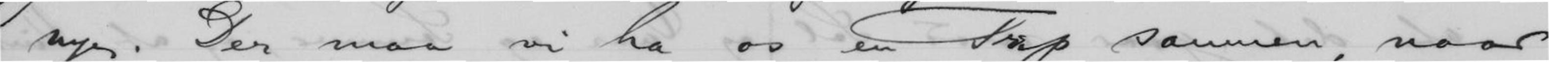nye. Der maa vi ha os en Trip sammen, naar
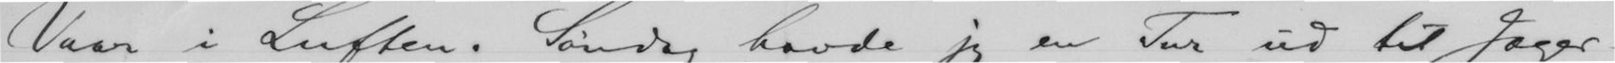Vaar i Luften. Søndag havde jeg en Tur ud til Jæger-
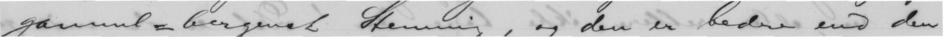gammel=bergensk Stemning, og den er bedre end den
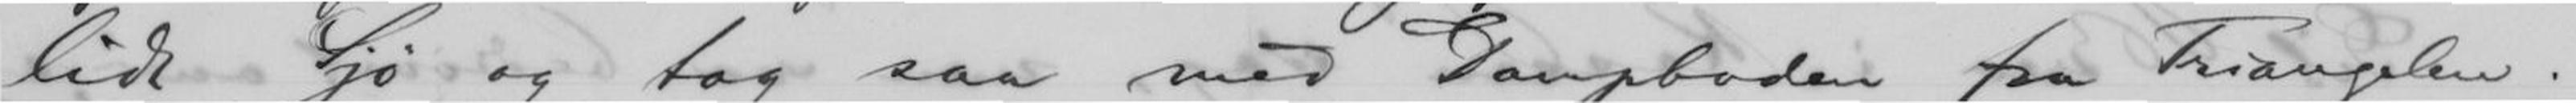lidt Sjø og tog saa med Dampboden fra Triangelen.
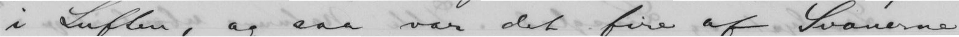i Luften, og saa var det fire af Svanerne
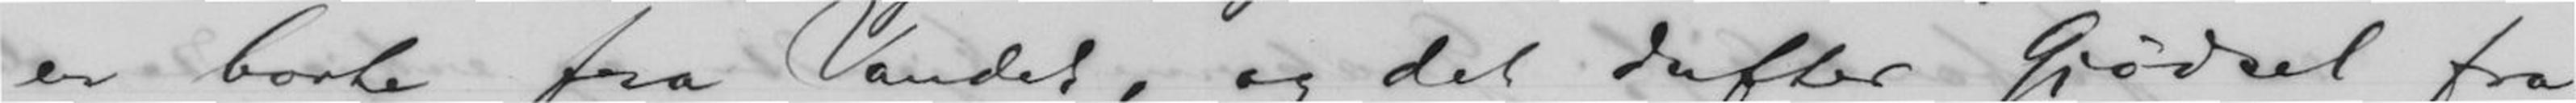er borte fra Vandet, og det dufter Gjødsel fra
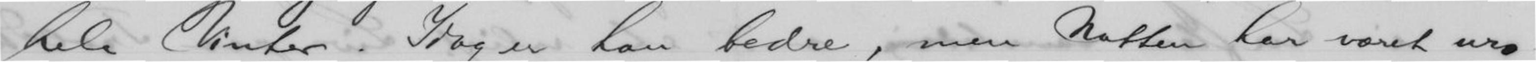hele Vinter. Idag er han bedre, men Natten har været uro-
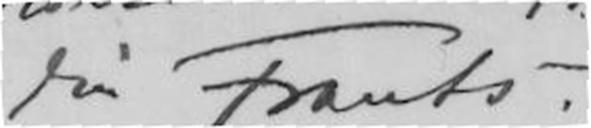Din Frants.
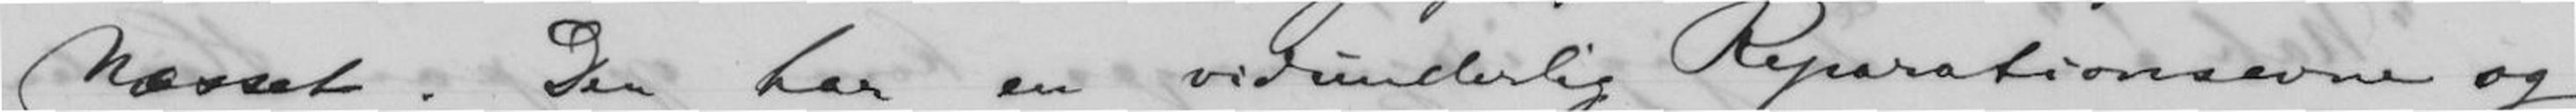Næsset. Den har en vidunderlig Reparationsevne og
